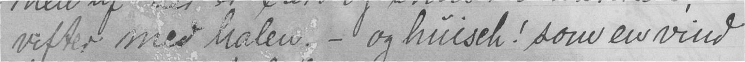vitter med halen;- og huisch! som en vind
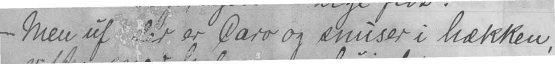- Men uf, der er Caro og snuser i hækken,
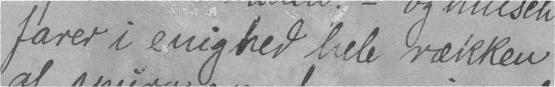farer i enighed hele rækken
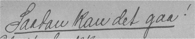Saadan kan det gaa!
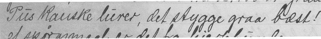Pus kanske lurer, det stygge graa bæst!
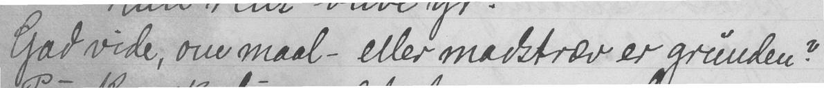Gud vide, om maal - eller madstræv er grunden?
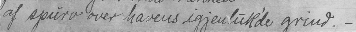af spurv over havens igjenluk'de grind. -
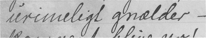urimeligt gnælder -
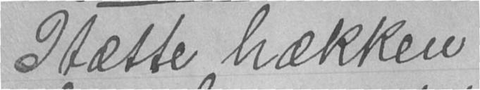I tætte hækken
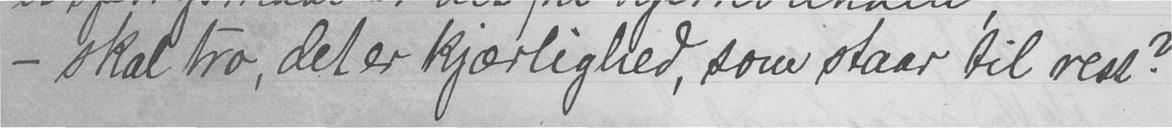- skal tro, det er kjærlighed, som staar til rest?
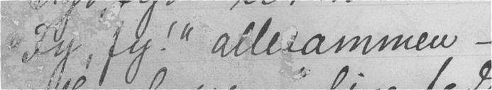"Fy, fy!" allesammen -
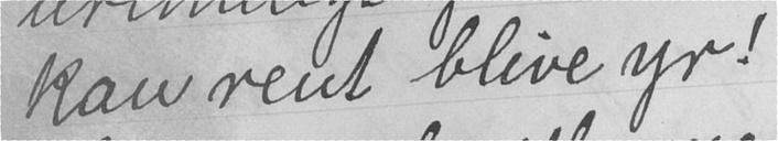kan rent blive yr!
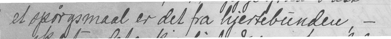et spørgsmaal er det fra hjertebunden, -
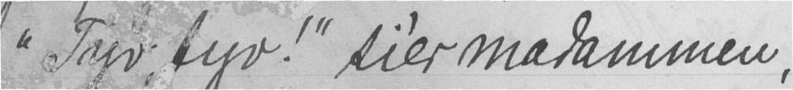"Tyv, tyv!" si'er madammen,
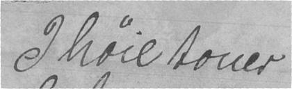I høie toner
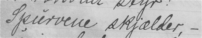Spurvene skjælder, -
